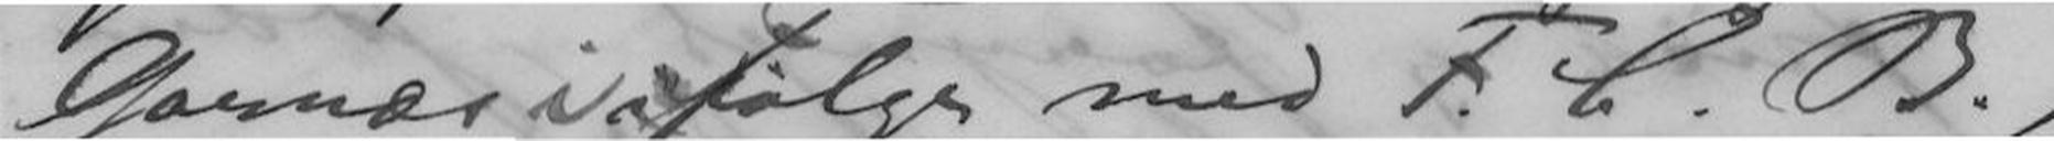Garnæs i følge med F.C.B.,
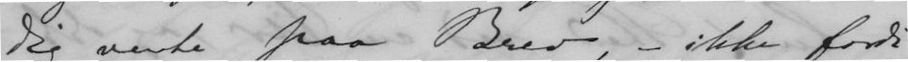Dig vente paa Brev, - ikke fordi
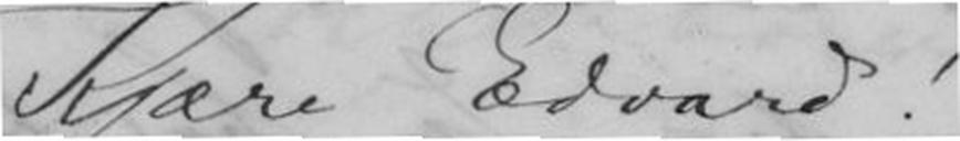Kjære Edvard!
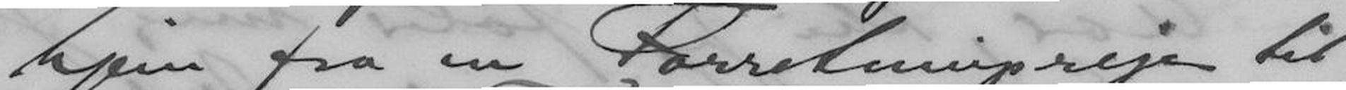hjem fra en Forretningsrejse til
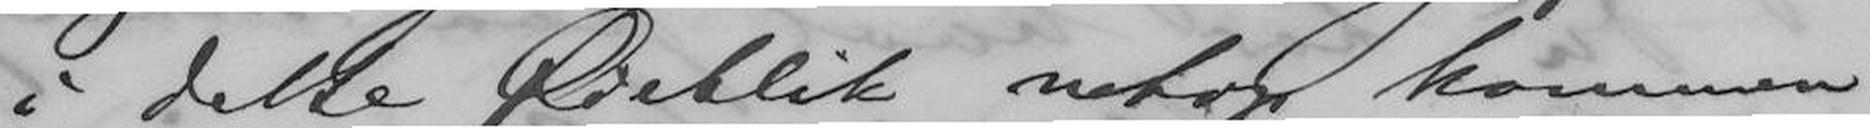i dette Øieblik netop kommen
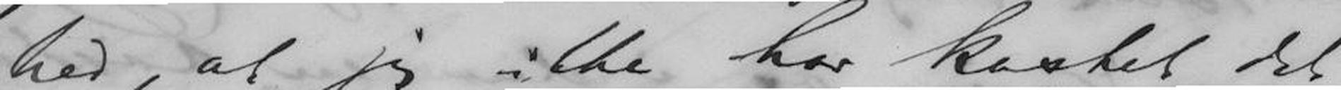hed, at jeg ikke har kastet det
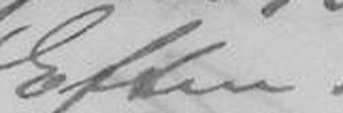Efterm.
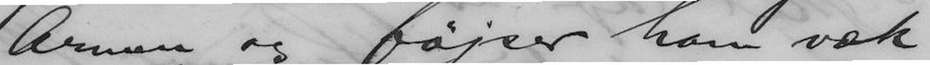Armen og føjser ham væk -
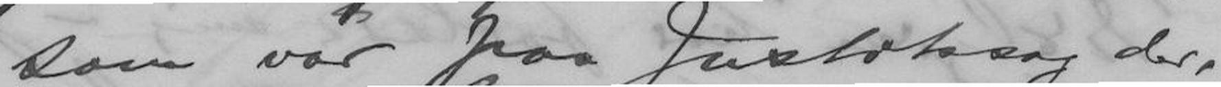som var paa Justitssag der,
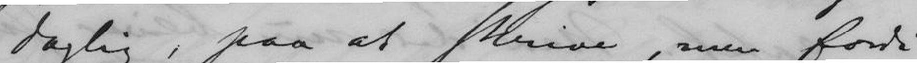daglig, paa at skrive, men fordi
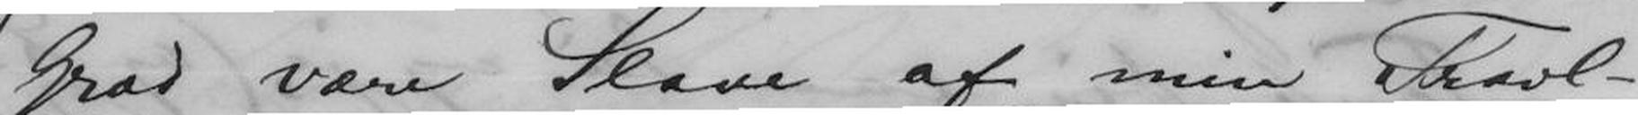Grad være Slave af min Travl-
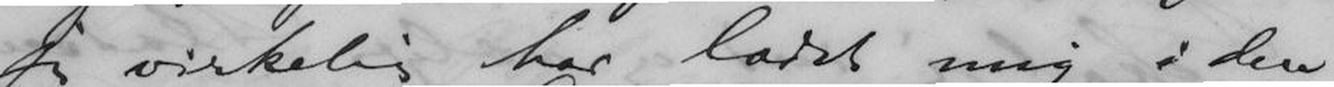jeg virkelig har ladet mig i den
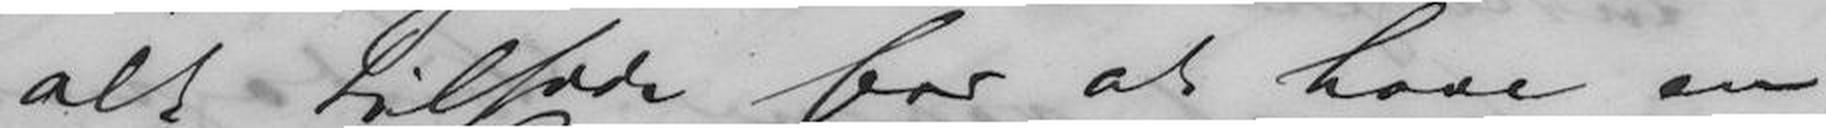alt tilside for at have en
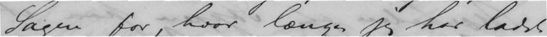Sagen for, hvor længe jeg har ladet
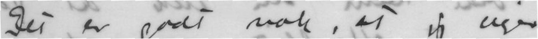Det er godt nok, at jeg siger
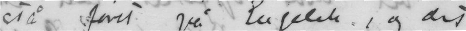stå først på Engelsk, og det
